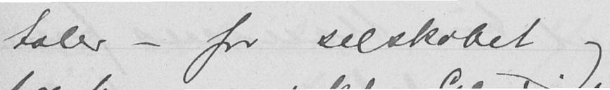taler - for selskabet og
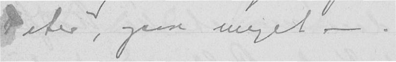Peter, ogsaa meget - .
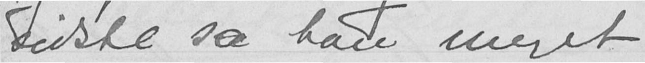sidste sa han meget
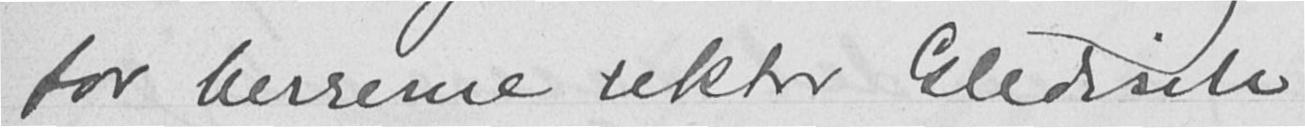for herrerne rektor Gledisch
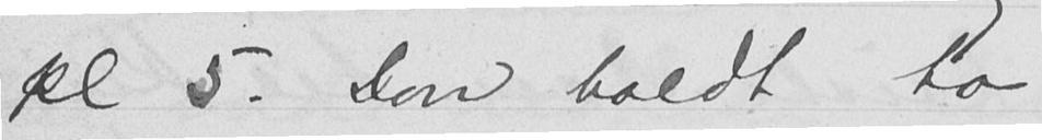kl 5. Don holdt to
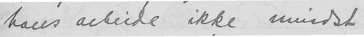hans arbeide ikke mindst
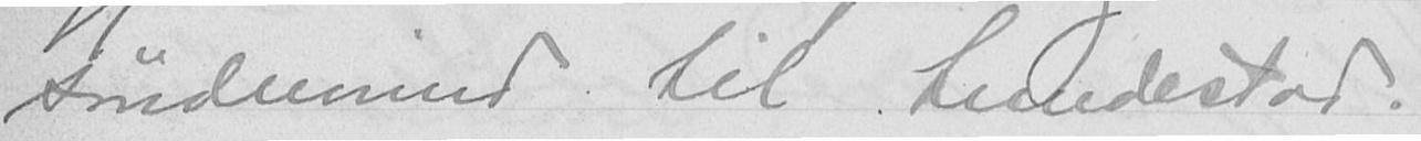søndenvind til Lundestad.
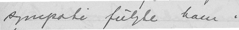sympati fulgte ham i
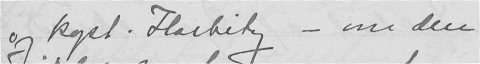og kapt. Harbitz - om den
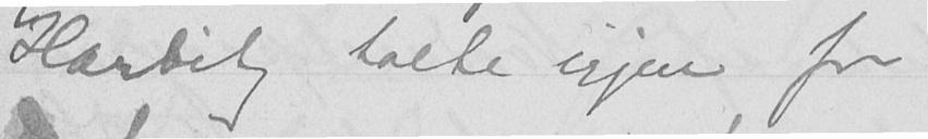Harbitz talte igjen for
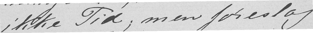ikke Tid, men foreslog
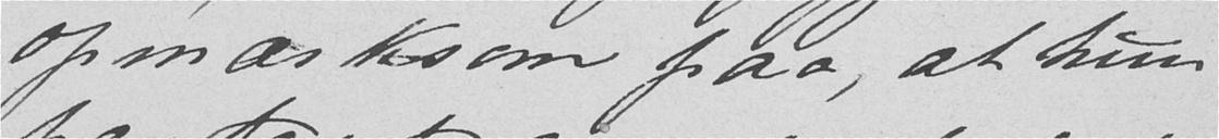opmærksom paa, at hun
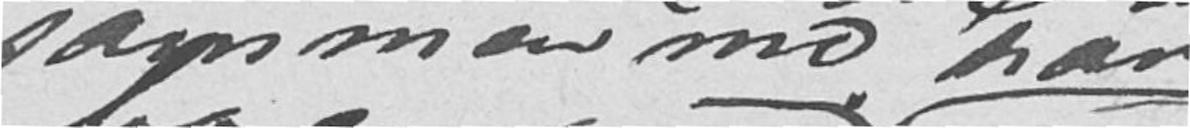sammen med ham
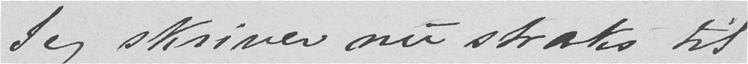Jeg skriver nu straks til
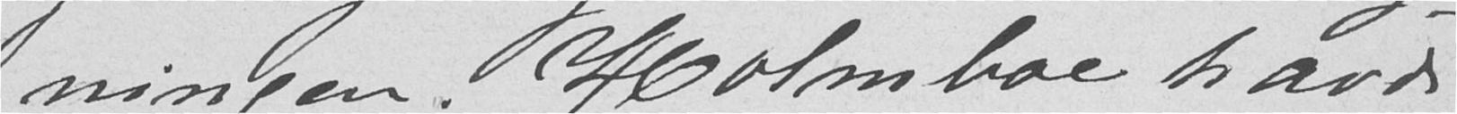ningen. Holmboe havde
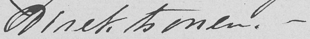Direktionen. -
